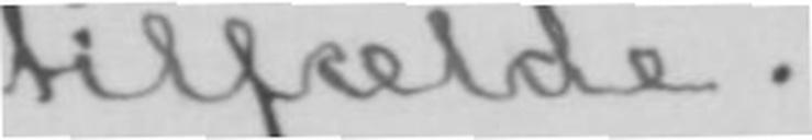tilfælde.
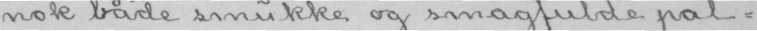nok både smukke og smagfulde pal-
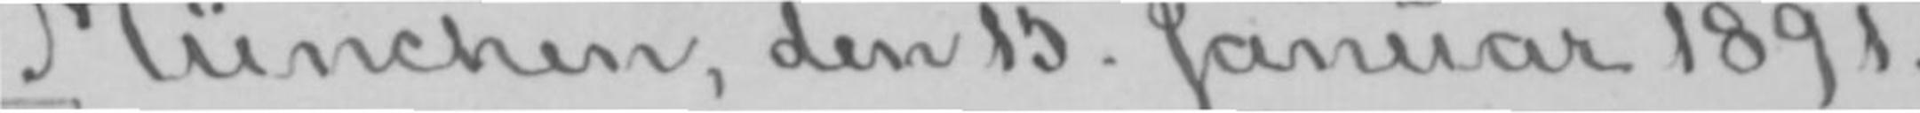München, den 15. Januar 1891.
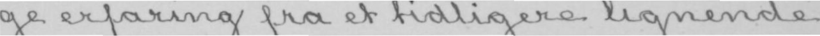ge erfaring fra et tidligere lignende
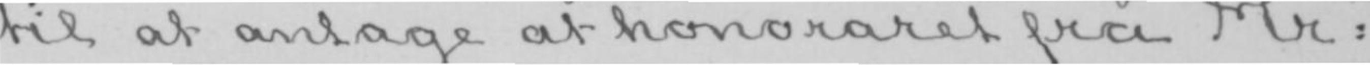til at antage at honoraret fra Mr:
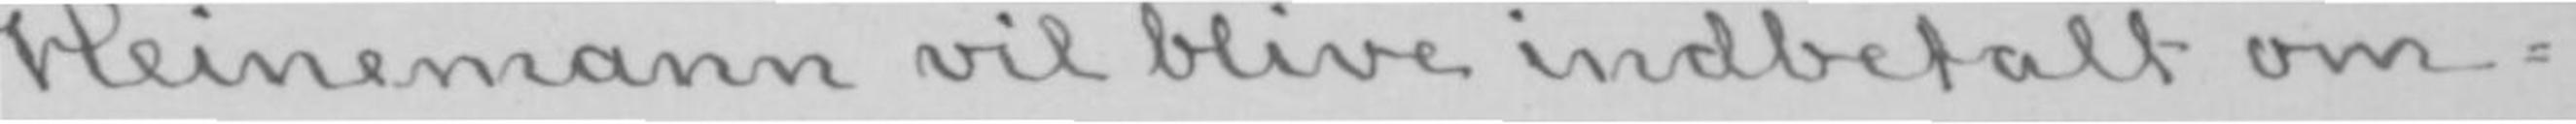Heinemann vil blive indbetalt om-
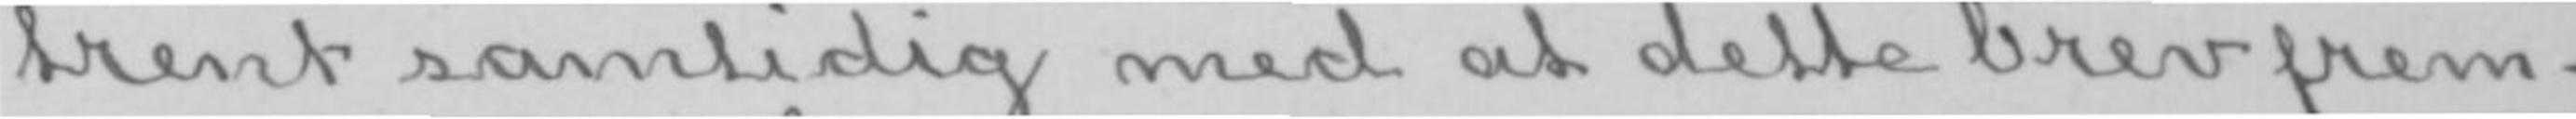trent samtidig med at dette brev frem-
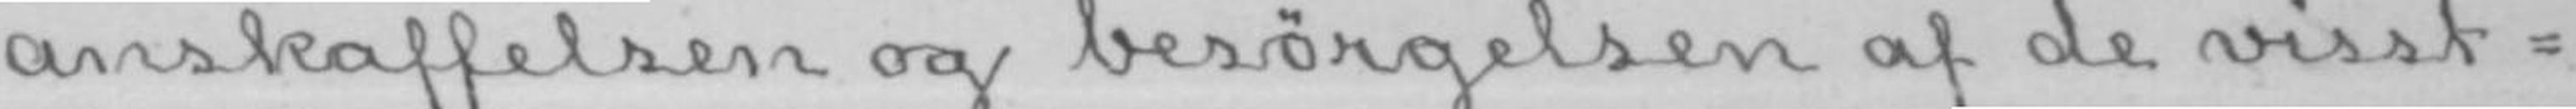anskaffelsen og besørgelsen af de visst-
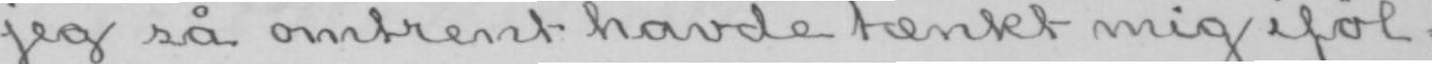jeg så omtrent havde tænkt mig iføl-
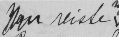reiste
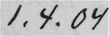1.4.04
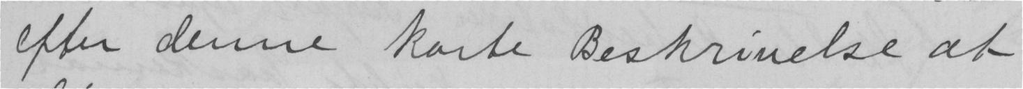efter denne korte Beskrivelse at
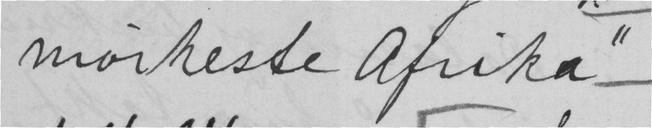mørkeste Afrika"
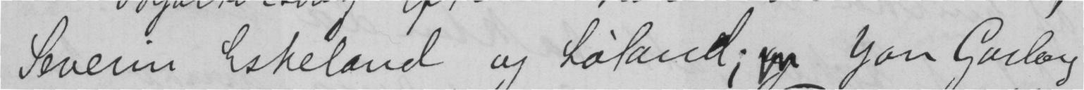Severin Eskeland og Løland;  Jon Garborg
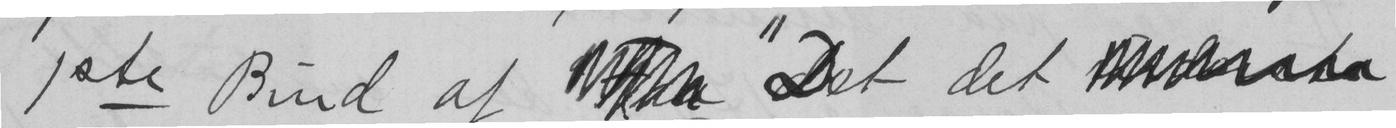1ste Bind af  "Det det
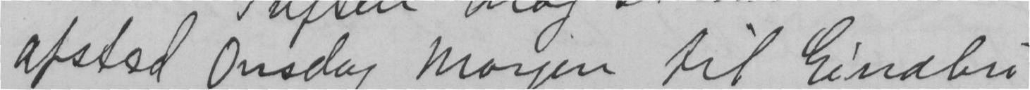afsted Onsdag morgen til Einabu
